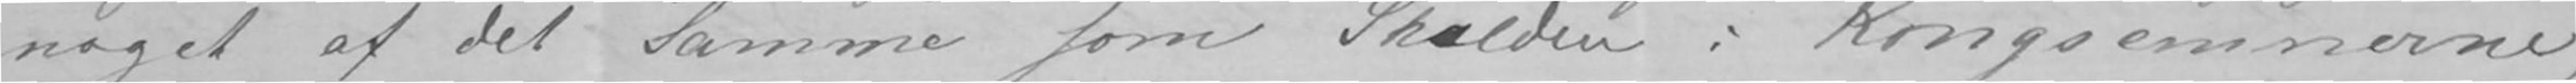noget af det Samme som Skalden i Kongsemnerne,
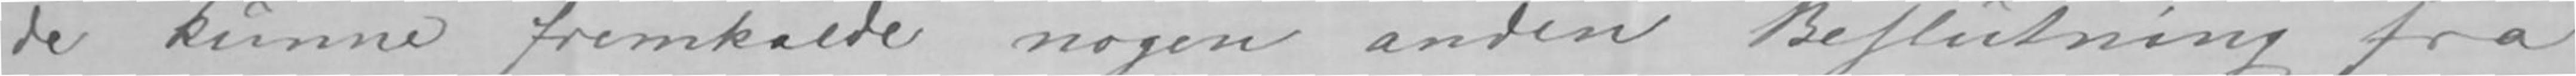de kunne fremkalde nogen anden Beslutning fra
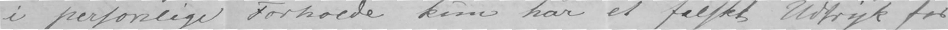i personlige Forholde kun har et falskt Udtryk for
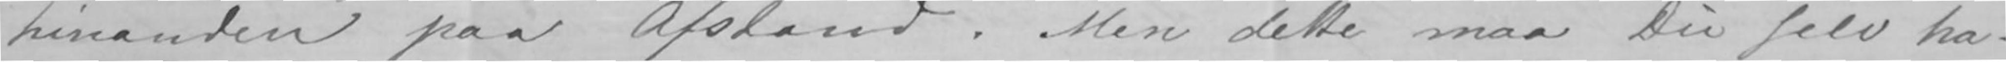hinanden paa Afstand. Men dette maa Du selv ha-
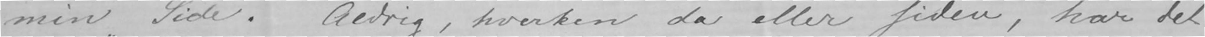min Side. Aldrig, hverken da eller siden, har det
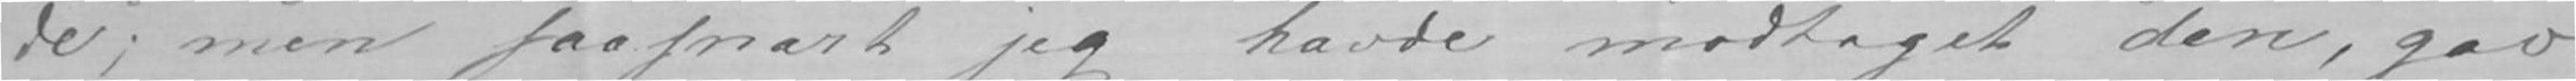de; men saasnart jeg havde modtaget den, gav
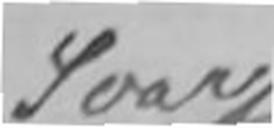Svar
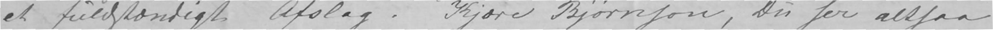et fuldstændigt Afslag. Kjære Bjørnson, Du ser altsaa
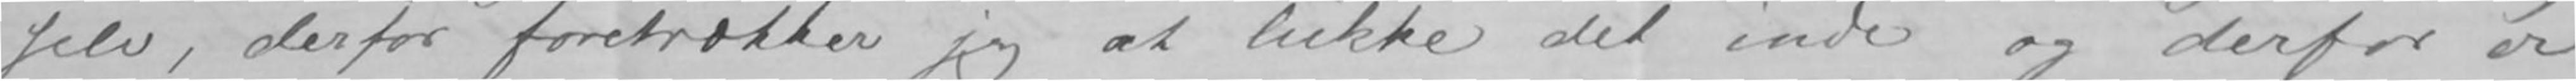selv, derfor foretrækker jeg at lukke det inde og derfor er
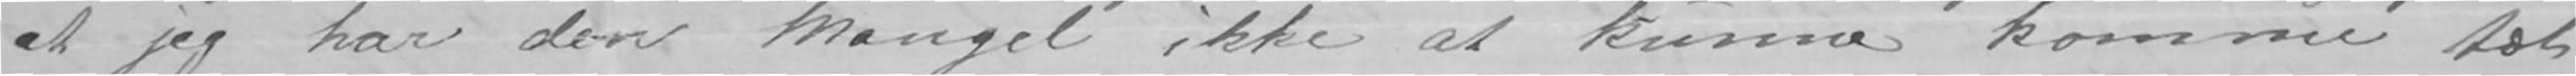at jeg har den Mangel ikke at kunne komme tæt
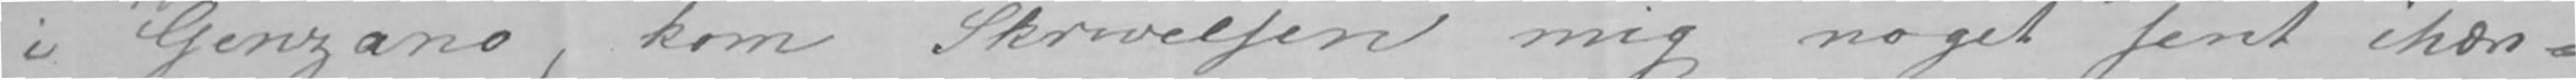i Genzano, kom Skrivelsen mig noget sent ihæn-
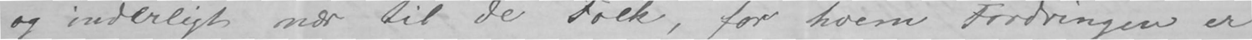og inderligt nær til de Folk, for hvem Fordringen er
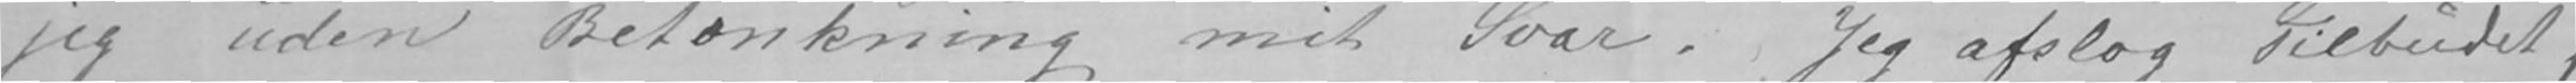jeg uden Betænkning mit Svar. Jeg afslog Tilbudet,
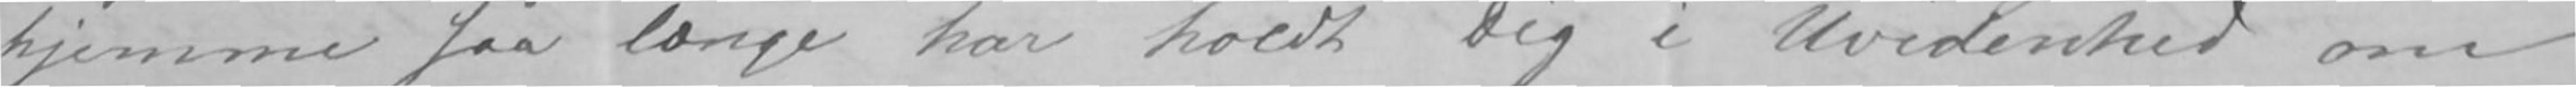hjemme saa længe har holdt Dig i Uvidenhed om
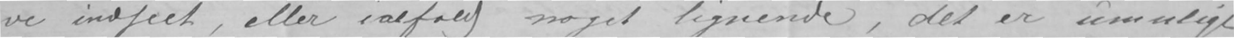ve indseet, eller ialfald noget lignende, det er umuligt
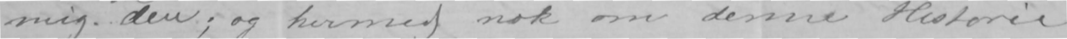mig den; og hermed nok om denne Historie.-
